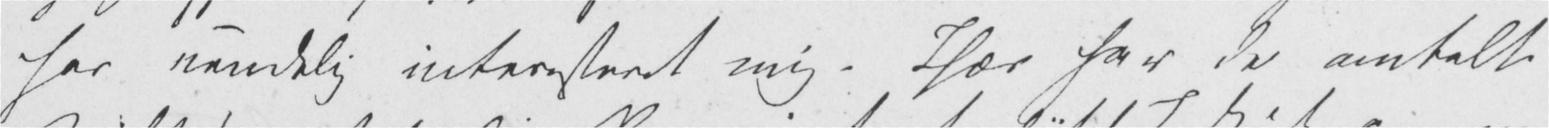har uendelig interesseret mig. Især har de omtalte
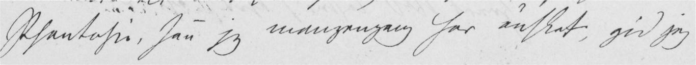Phantasie, saa jeg mangengang har ønsket, gid jeg
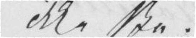ikke ske.
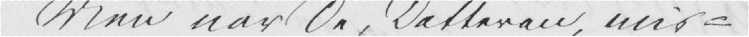Men når De, Datteren, mis¬
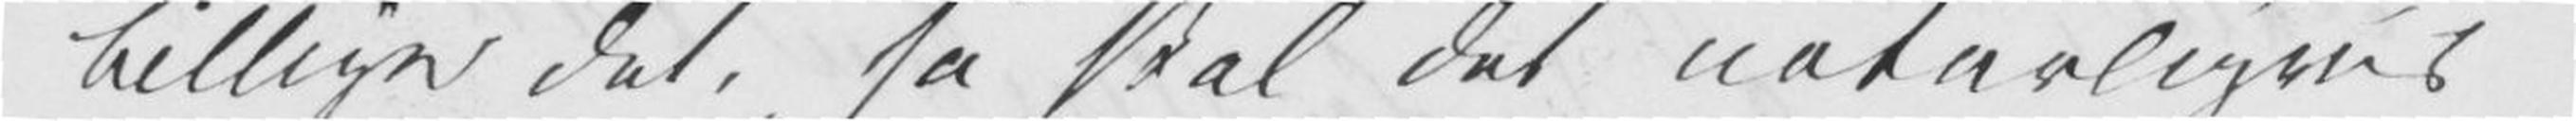billiger det, så skal det naturligvis
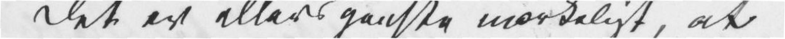Det er ellers ganske mærkeligt, at
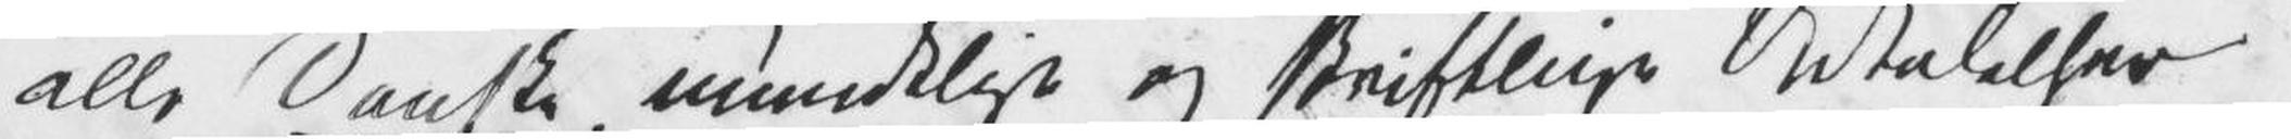alle Danske, mundtlige og skriftlige Udtalelser
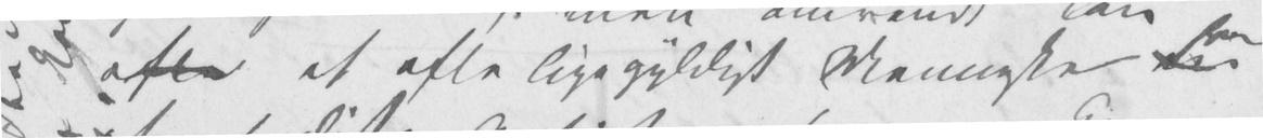ofte et ofte ligegyldigt Menneske eie have
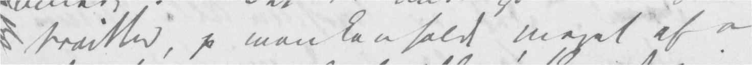traitter, ja man kan holde meget af
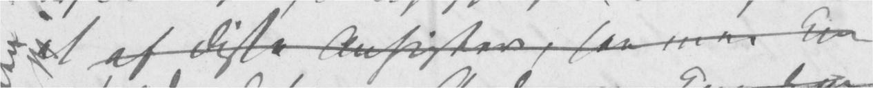et af disse Ansigter, saa man kan
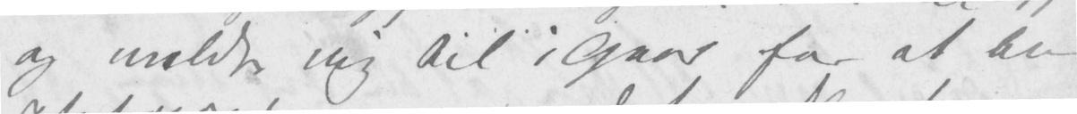og meldte mig til i Gaar for at bl
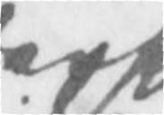derfor
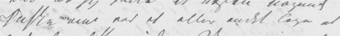Ønske om ved et eller andet Tegn at
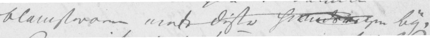blomsterarme disse smuds By,
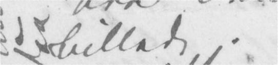Billede.
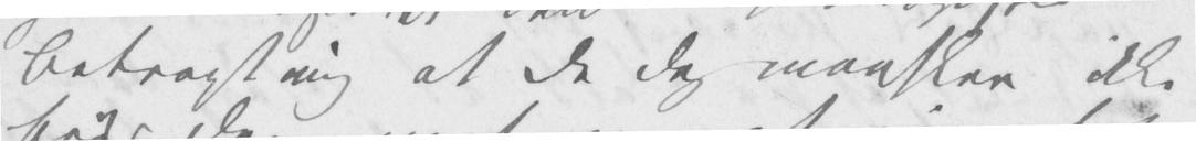Betragtning at De dog maaskee ikke
